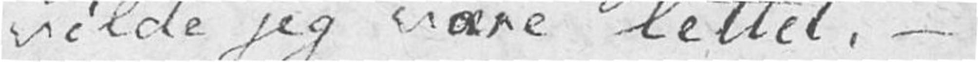vilde jeg være lettet. –
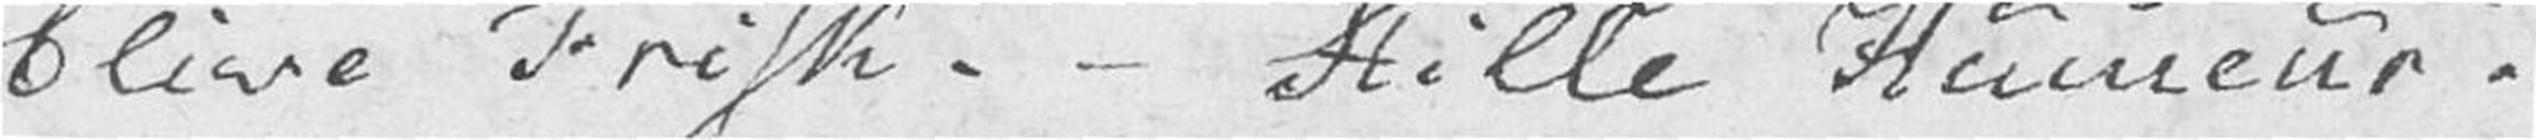blive Frisk. – Stille Humeur.
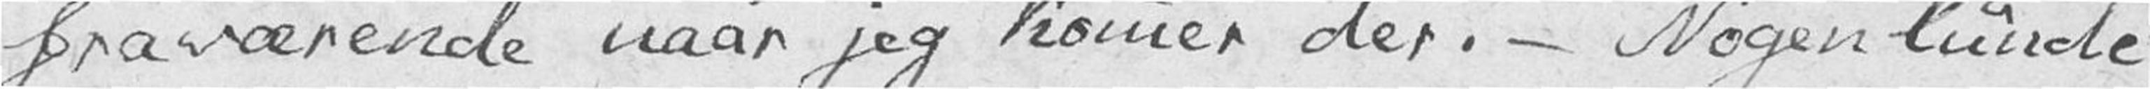fraværende naar jeg kommer der. – Nogenlunde
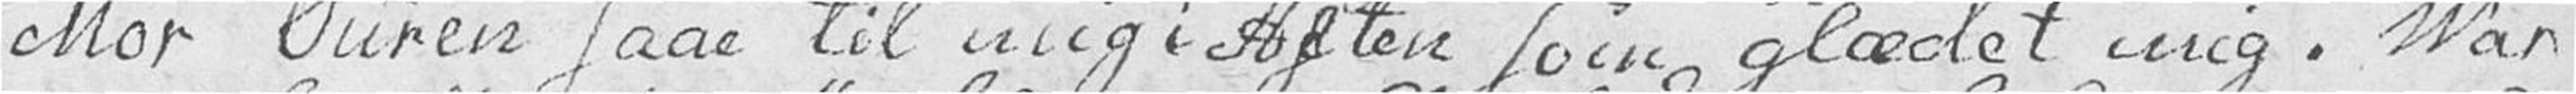Mor Ouren saae til mig i Aften som glædet mig. Var
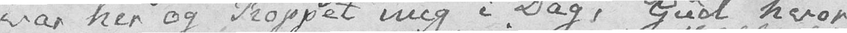var her og Koppet mig i Dag, Gud hvor
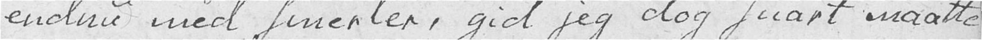endnu med smerter, gid jeg dog snart maatte
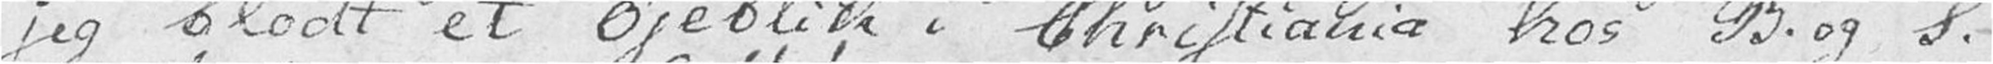jeg blodt et Øjeblik i Christiania hos B. og S.
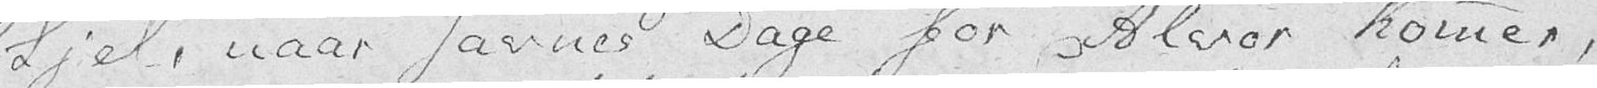Sjel, naar savnes Dage for Alvor kommer,
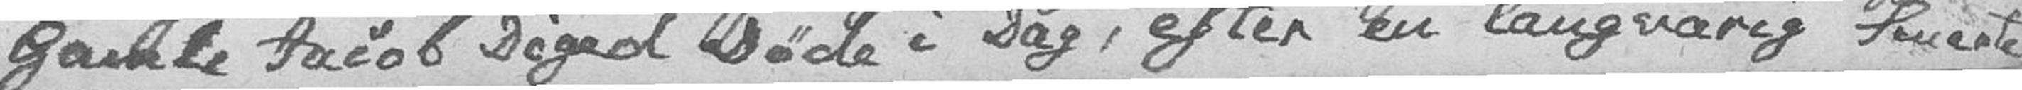Gamle Jacob Diged Døde i Dag, efter en langvarig Smerte
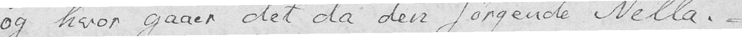og hvor gaaer det da den sørgende Nella. –
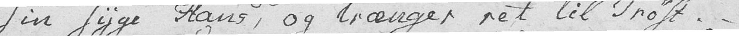sin syge Hans, og trænger ret til Trøst. –
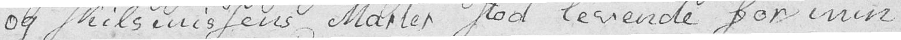og skilsmissens Marter stod levende for min
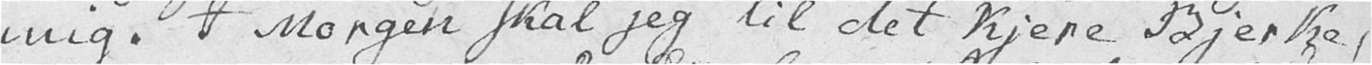mig. I Morgen skal jeg til det kjere Bjerke,
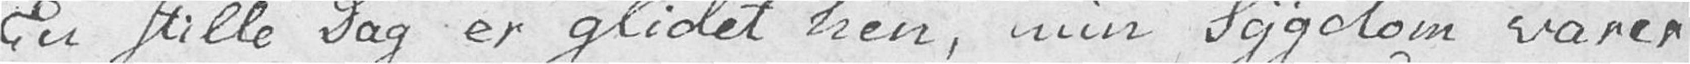En stille Dag er glidet hen, min Sygdom varer
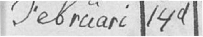Februari 14d
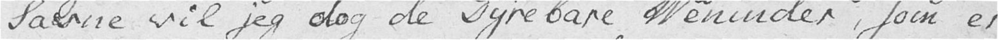Savne vil jeg dog de Dyrebare Veninder, som er
